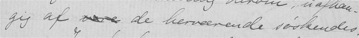gig af vore de herværende søskendes.
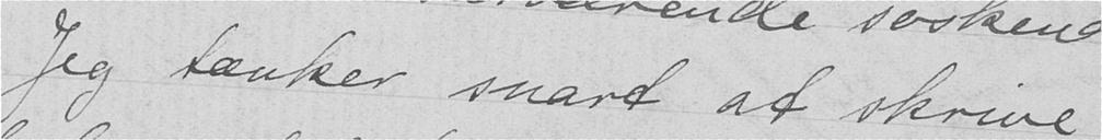Jeg tænker snart at skrive
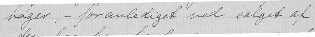bøger, - foranlediget ved salget af
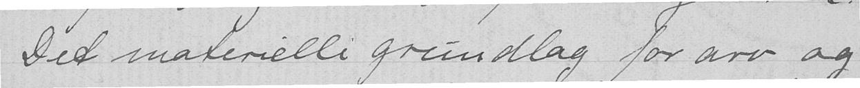"Det materielle grundlag for arv og
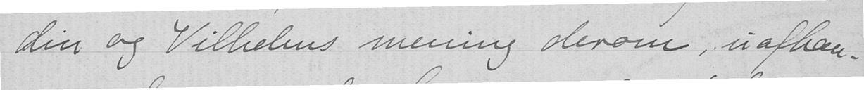din og Vilhelms mening derom, uafhæn-
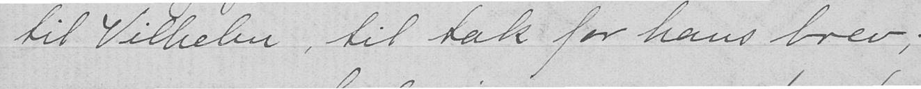til Vilhelm, til tak for hans brev;
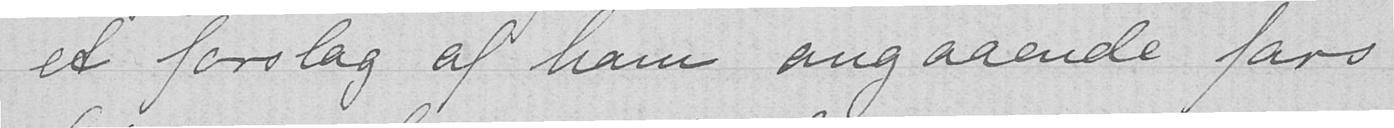et forslag af ham angaaende fars
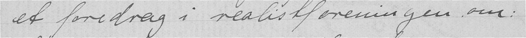et foredrag i realistforeningen, om:
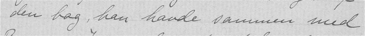den bog, han havde sammen med
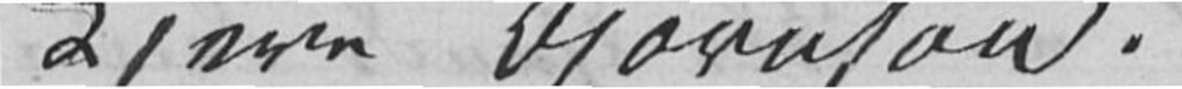Kjere Bjørnson.
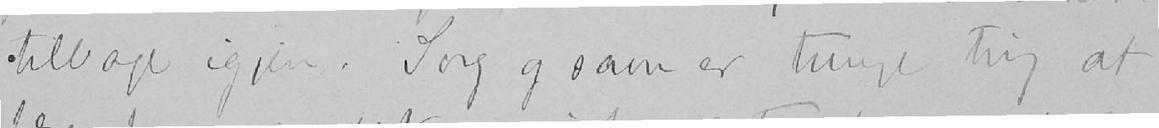tilbage igjen. Sorg og savn er tunge ting at
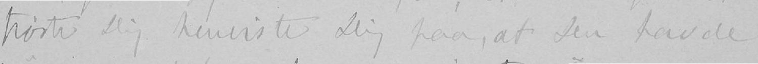trøste Dig henviste Dig paa, at Du havde
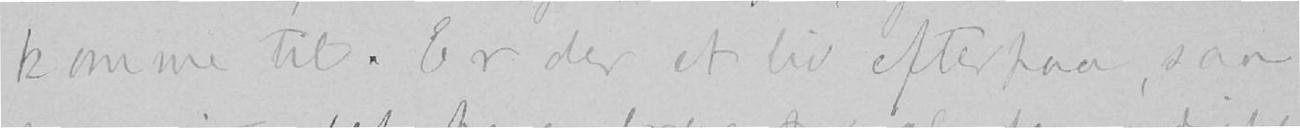komme til. Er der et liv efterpaa, saa
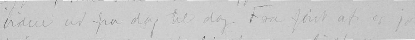videre ud fra dag til dag. Fra først af er jo
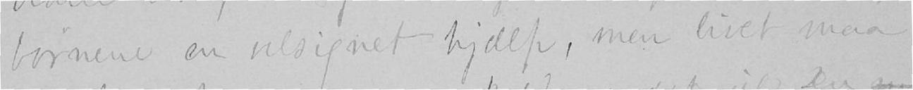børnene en velsignet hjælp, men livet maa
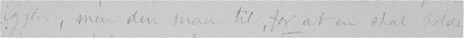igjen, men den maa til, for at en skal holde
